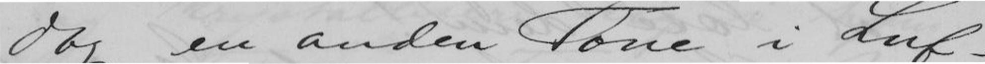dog en anden Tone i Luf-
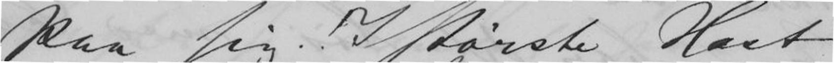paa sig. I største Hast,
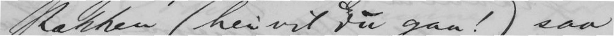stakken (hei vil du gaa!) saa
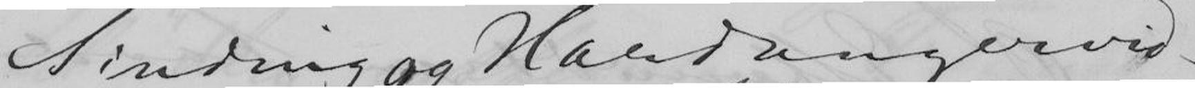Sinding og Hardangervid-
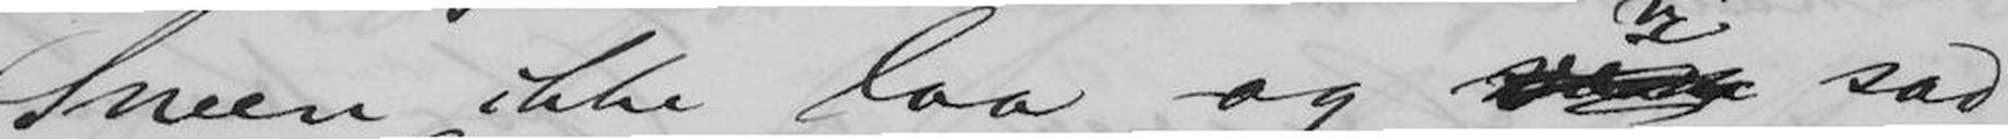Sneen ikke laa og vi vis sad
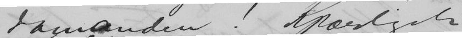damanden! Kjærligste
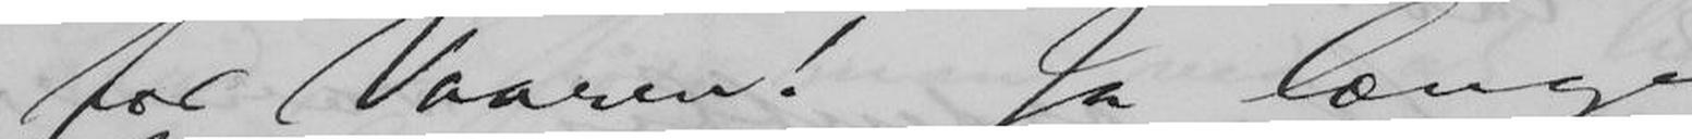for Vaaren! Ja længe
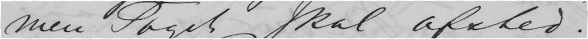men Toget skal afsted.
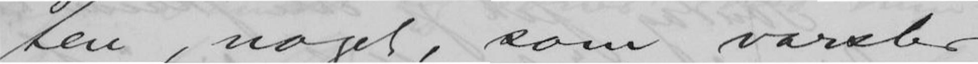ten, noget, som varsler
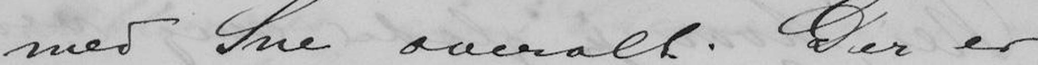med Sne overalt. Der er
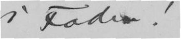i Foden!
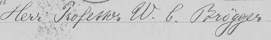Herr Professor W. C. Brøgger
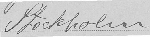Stockholm
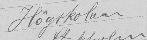Høgskolan
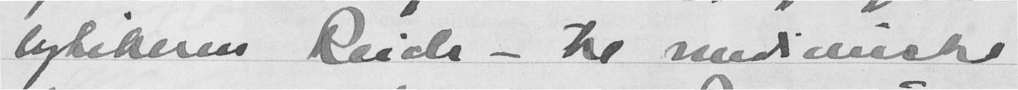lytikeren Reich - tre medicinske
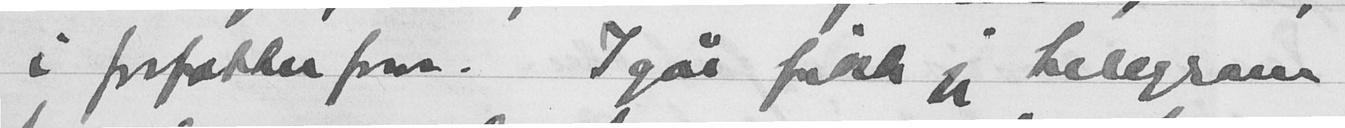i forfatterforn. Igår fikk jeg telegram
